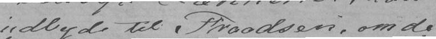ndbyde til Fraadsen, om de
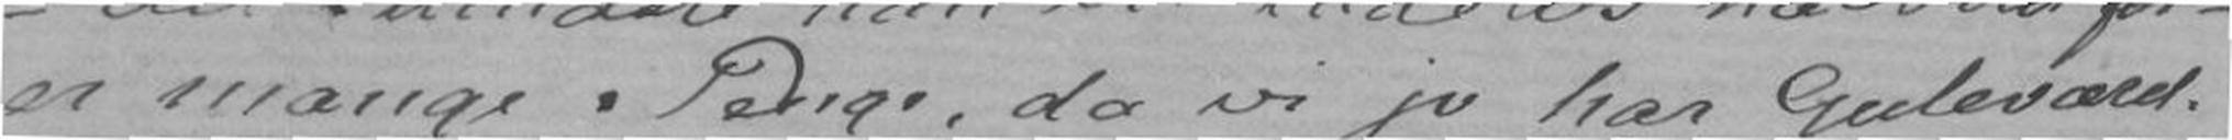er mange Penge, da vi jo har Guleværel-
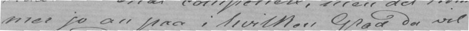mer jo an paa i hvilken Grad Du vil
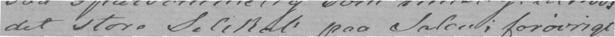det store Selskab paa Salen; forøvrigt
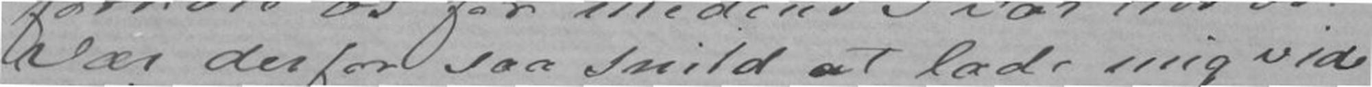Vær derfor saa snild at lade mig vide
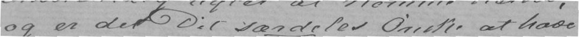og er det Dit særdeles Ønske at have
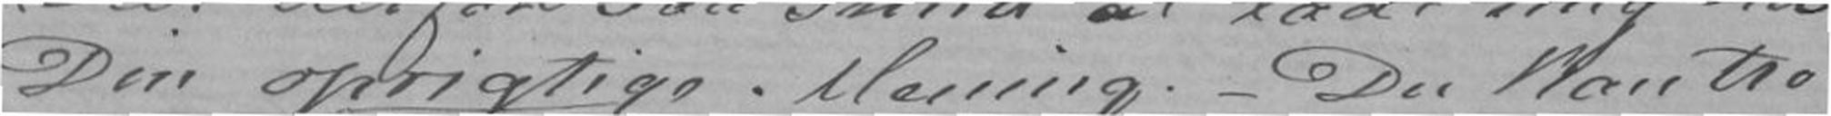Din oprigtige Mening. - Du kan tro
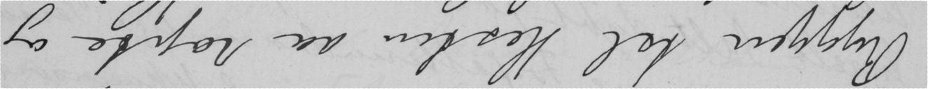Ryggen tel Hesten aa ropte og
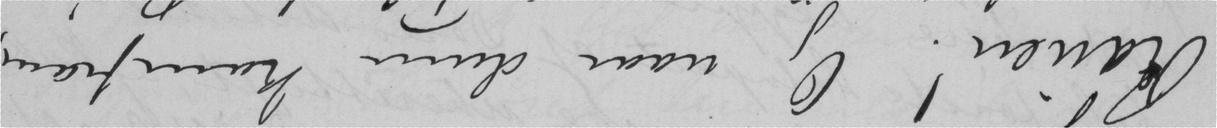Rauen! Og naar dum kom fram,
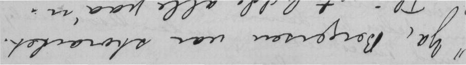"Ja, Beyersen var storartet.
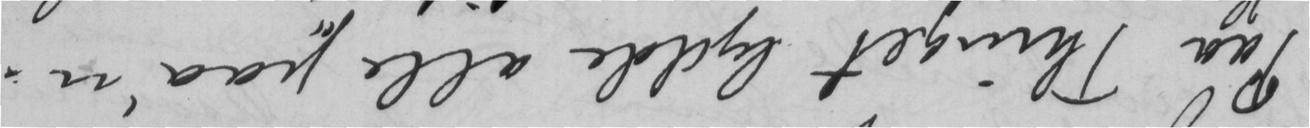Paa Thinget lydde alle paa'n.
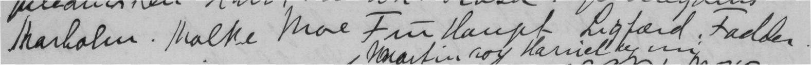Marholm. Molke Moe Fru Haupt Ligfærd. Fadder.
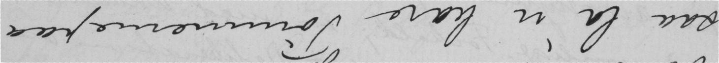saa la'n bare Tømmerne paa
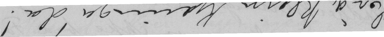Er'a klein Kjerringa da!
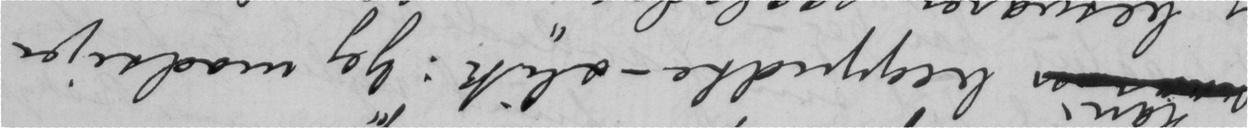begyndte slik: "Jeg modsiger
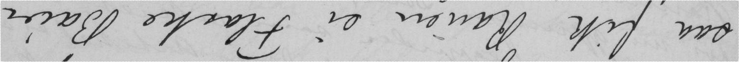saa fik Rauen ei Flaske Baier
